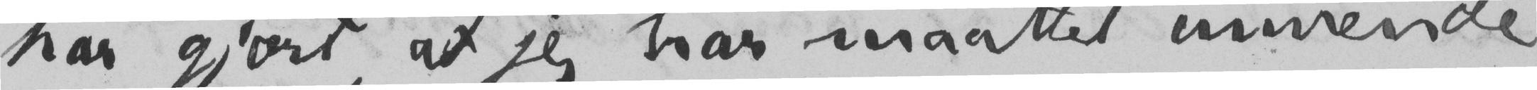har gjort, at jeg har maattet anvende
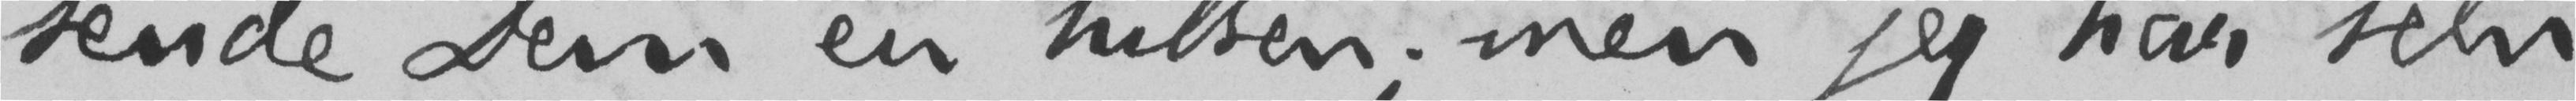sende Dem en hilsen; men jeg har selv
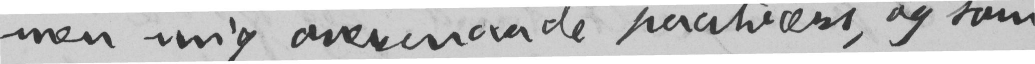men mig overmaade paatværs, og som
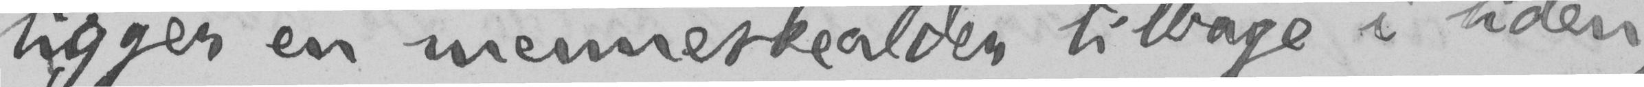ligger en menneskealder tilbage i tiden,
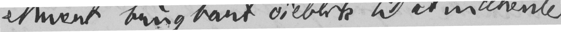ethvert brugbart øieblik til at indhente
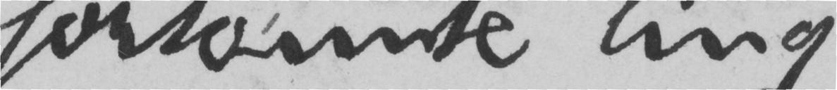forsømte ting.
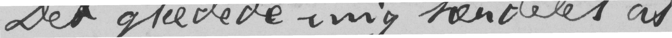Det glædede mig særdeles at
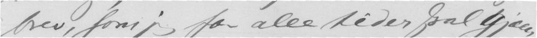brev, som jeg for alle tider skal gjæm-
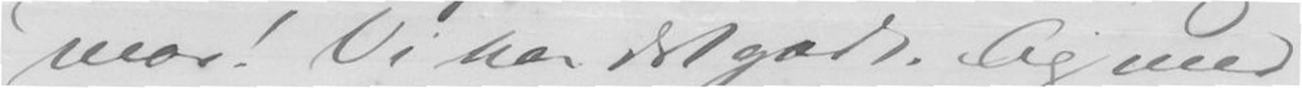mor! Vi har det godt. Og med
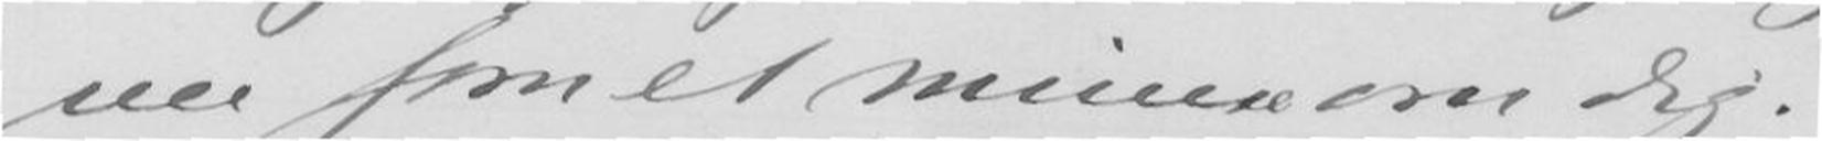me som et minne om dig.
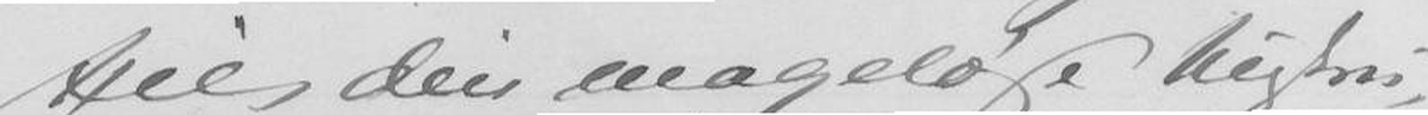Hils din mageløse hustru,
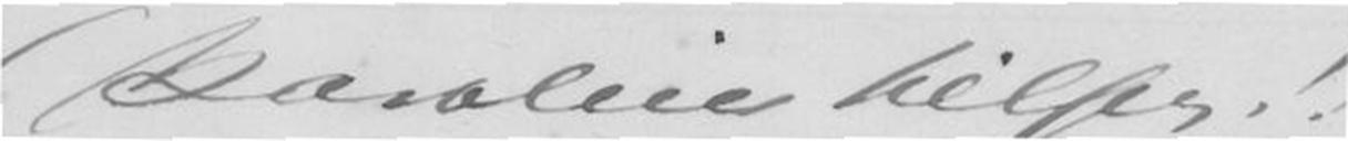(Karoline hilser!!)
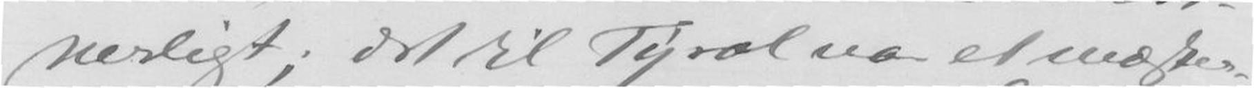nerligt; det til Tyrol var et mæster-
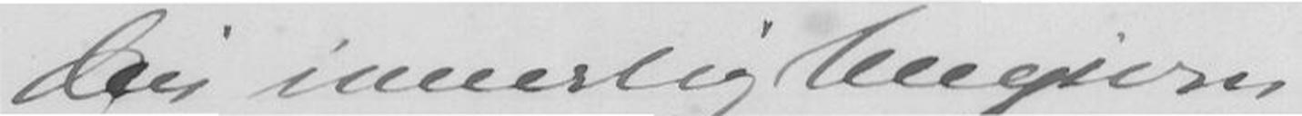din innerlig hengivne
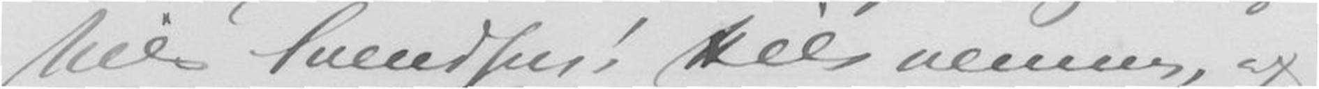hils Svendsen! Hils venner, og
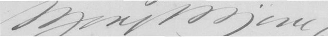Bjørnst Bjørnson
